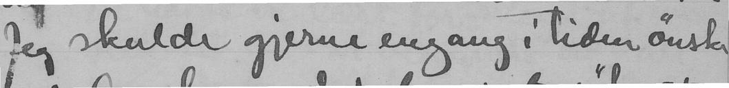Jeg skulde gjerne engang i tiden ønske
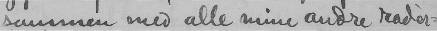sammen med alle mine andre rader-
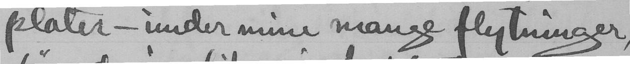plater - under mine mange flytninger,
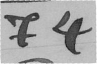74
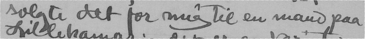solgte det for mig til en mand paa
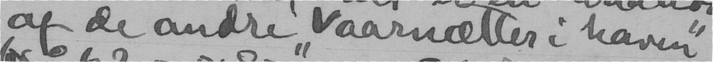af de andre "vaarnætter i haven"
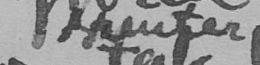enten
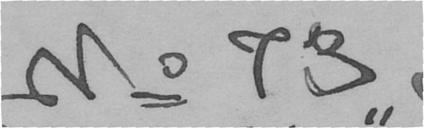No 73
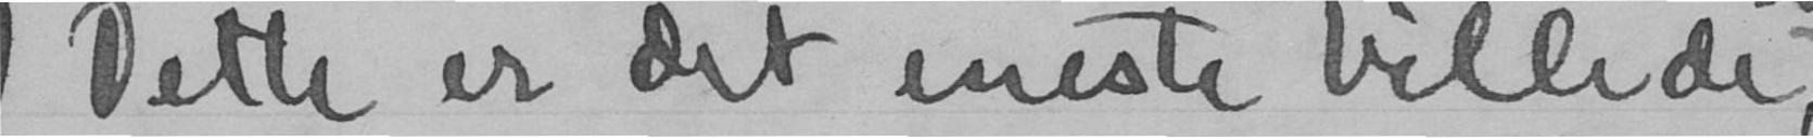Dette er det eneste billede
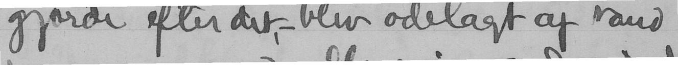gjorde efter det, blev ødelagt af vand
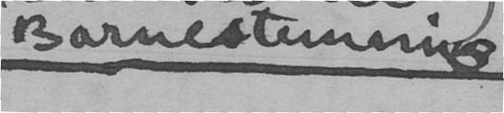Barnestemning
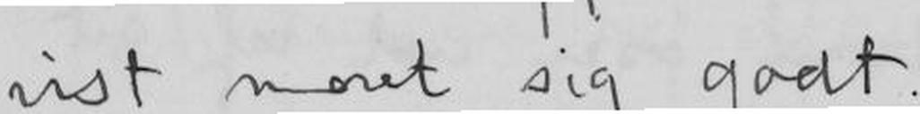vist moret sig godt.
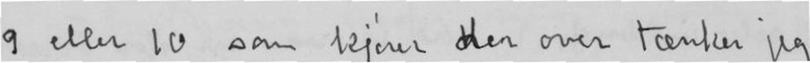9 eller 10 som kjører der over Tænker jeg.
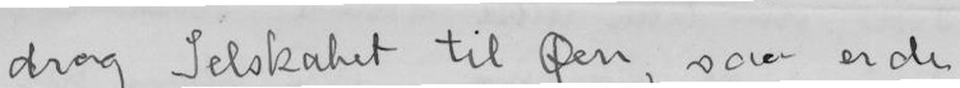drog Selskabet til Øen, saa er de
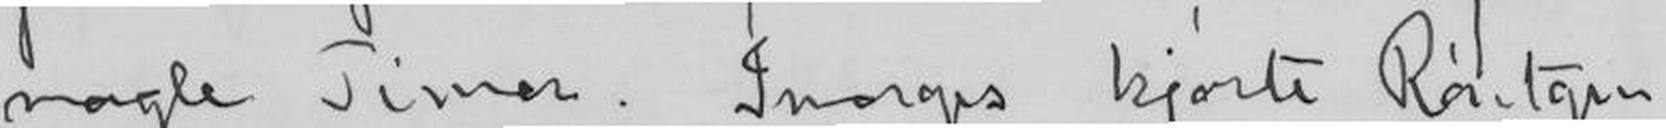nogle Timer. Imorges kjørte Røntgen
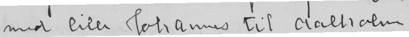med lille Johannes til Malholm,
